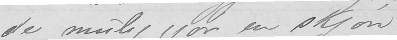de muliggjør en skjøn
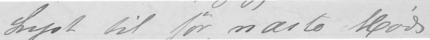Lyst til før næste Møde
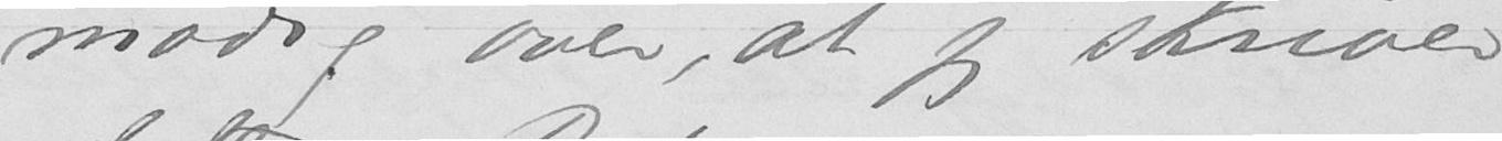modig over, at jeg skriver
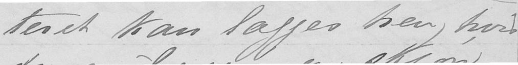teret kan lægges hen, hvis
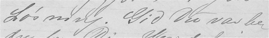Løsning. Gid Du var be-
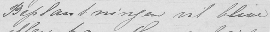Beplantningen vil blive
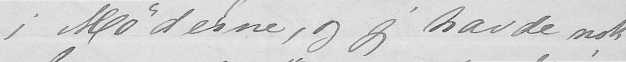i Møderne, og jeg havde nok
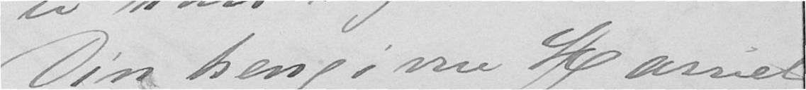Din hengivne Harriet
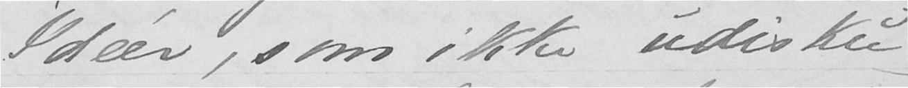Idéer, som ikke udisku-
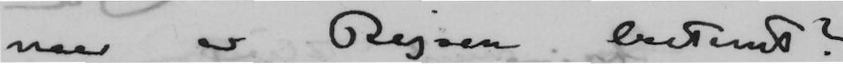naar er Rejsen bestemt?
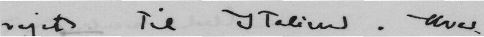rejst til Italien. Hvad
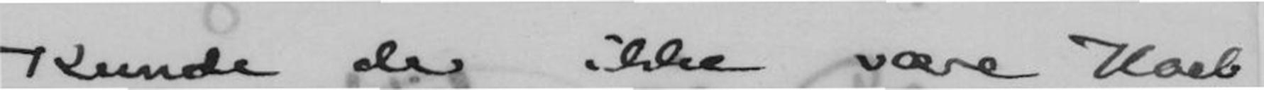Kunde det ikke være Haab
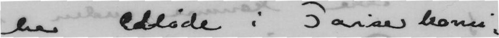har Møde i Garisekommi-
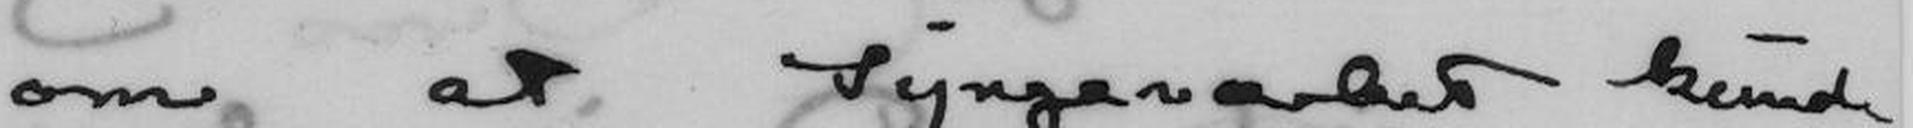om at Syngeværket kunde
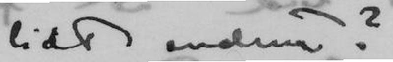lidt endnu ?
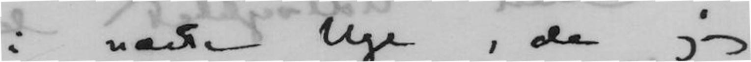i næste Uge, da jeg
